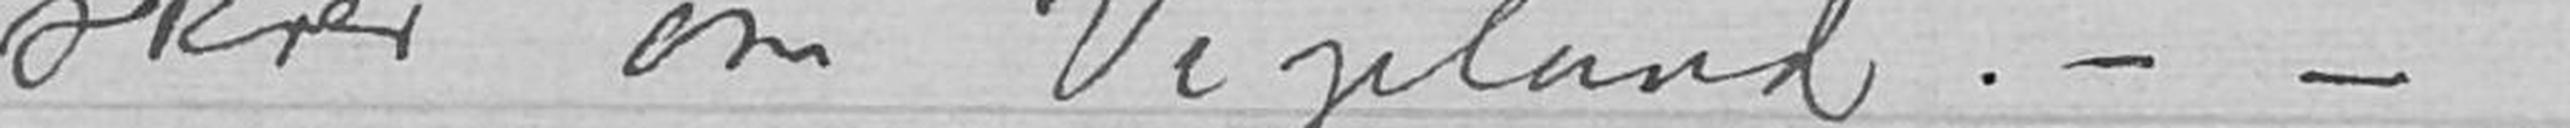skrev om Vigeland. – –
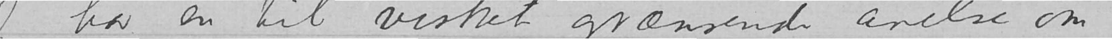Jeg har en til vishet grænsende anelse om,
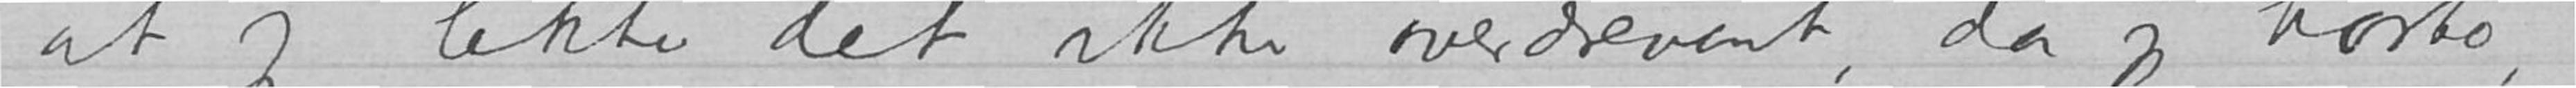at jeg likte det ikke overdrevent, da jeg hørte,
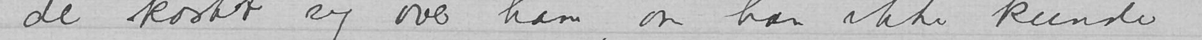de kastet sig over ham, om han ikke kunde
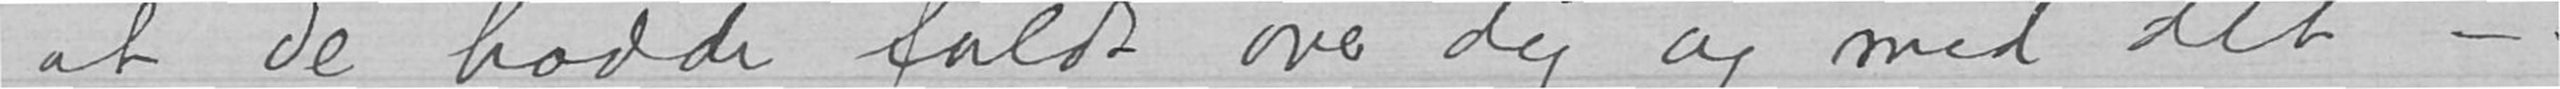at de hadde faldt over dig og med det –
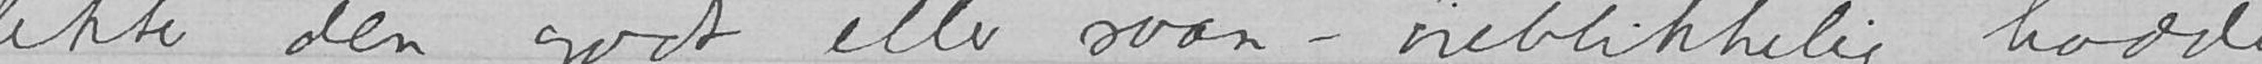likte den godt eller saan – øieblikkelig hadde
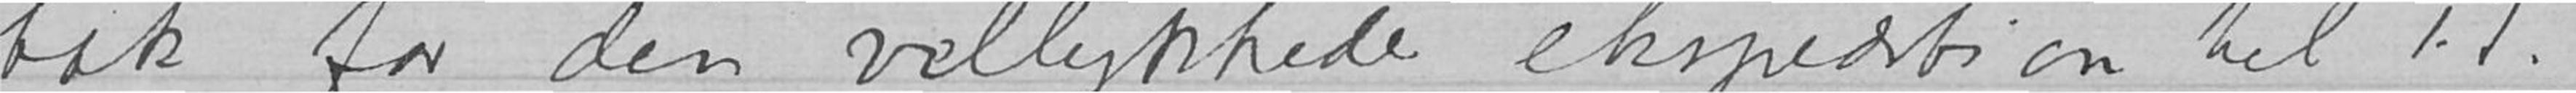tak for din vellykkede ekspedition til T.T.
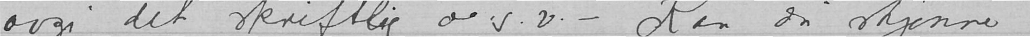avgi det skriftlig o.s.v. – Kan du skjønne
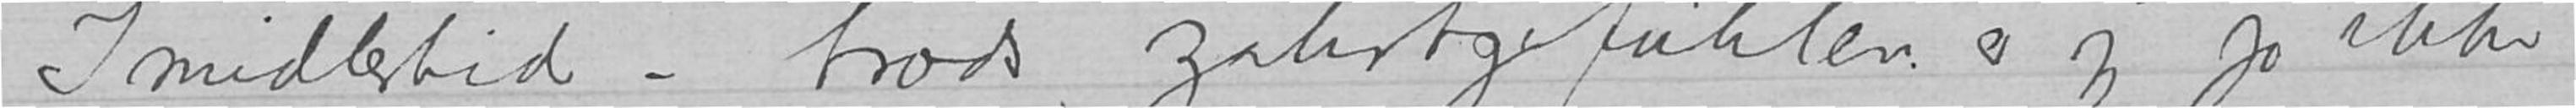Imidlertid – trods zahrtgefühlen, er jeg jo ikke
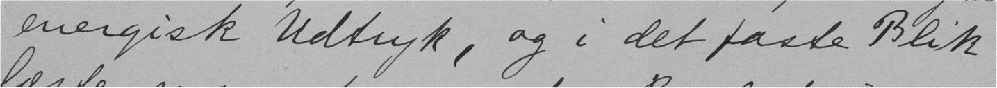energisk Udtryk, og i det faste Blik
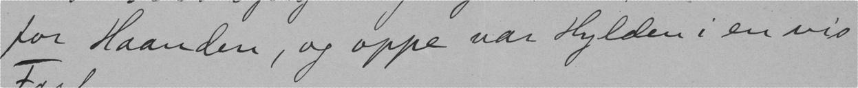for Haanden, og oppe var Hylden i en vis
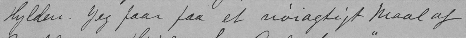Hylden. Jeg faar faa et nøiagtigt Maal af
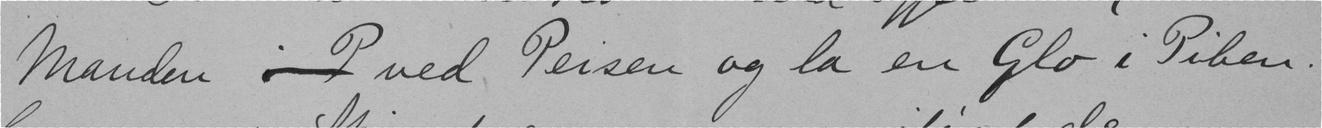Manden i P ved Peisen og la en Glo i Piben.
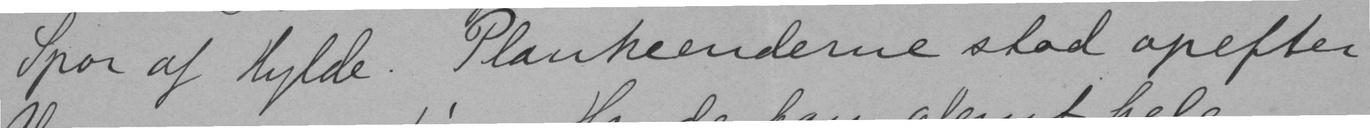Spor af Hylde. Plankeenderne stod opefter
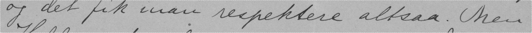og det fik man respektere altsaa. Men
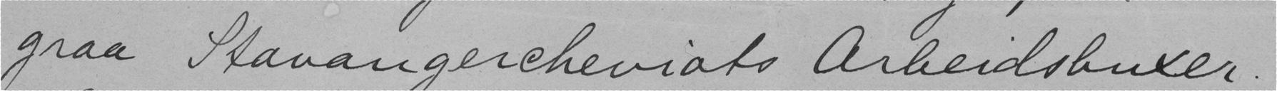graa Stavangercheviats Arbeidsbuxer.
